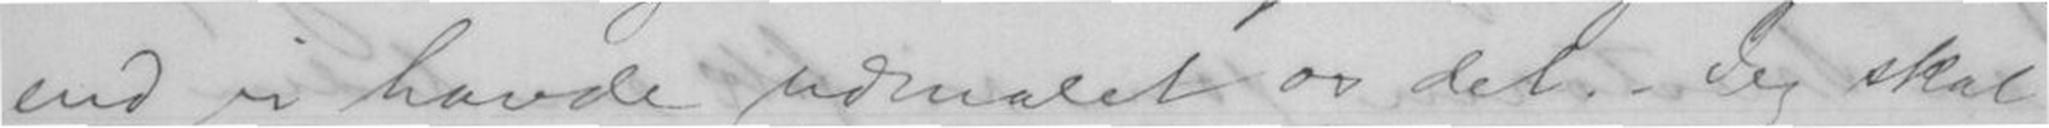end vi havde udmalet os det. - Jeg skal
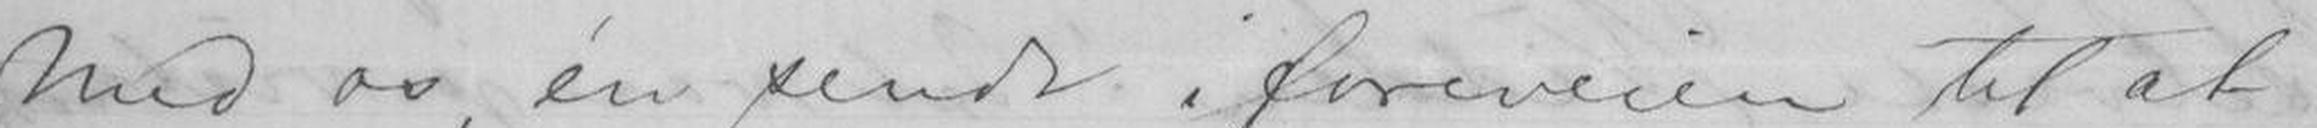med os, én sendt iforveien til at
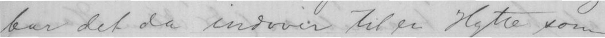bar det da indover til en Hytte som
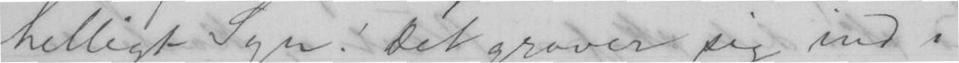helligt Syn! Det graver sig ind i
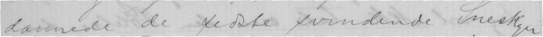dannede de sidste svindende Sneskyer
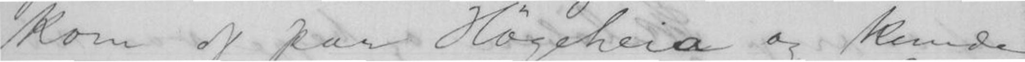kom af paa Høgeheia og kunde
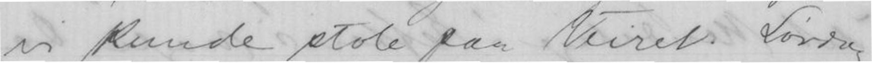vi kunde stole paa Veiret. Lørdag
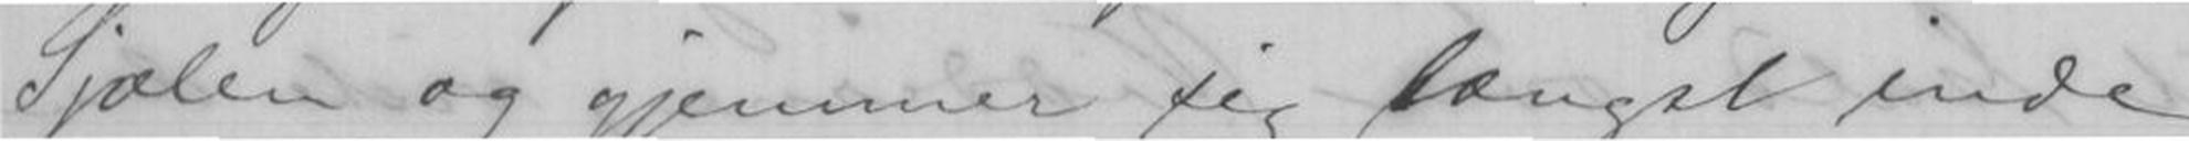Sjælen og gjemmer sig længst inde
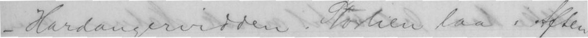- Hardangervidden. Storlien laa i Aften
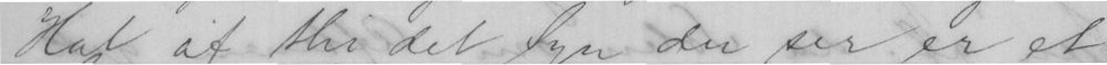Hat af thi det Syn du ser er et
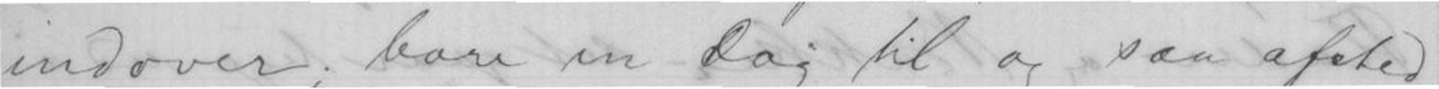indover; bare en Dag til og saa afsted,
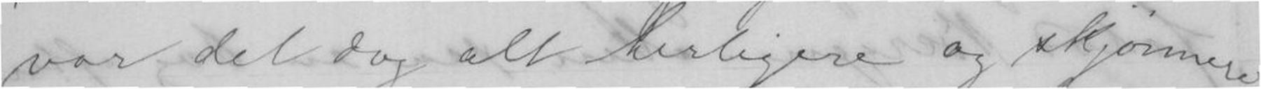var det dog alt herligere og skjønnere
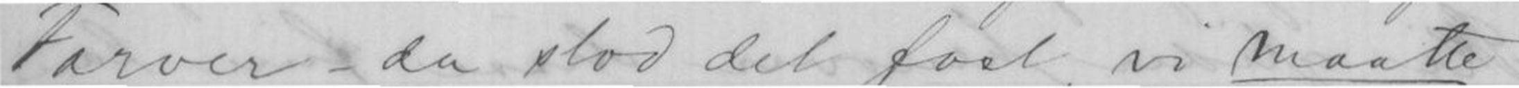Farver - da stod det fast, vi maatte
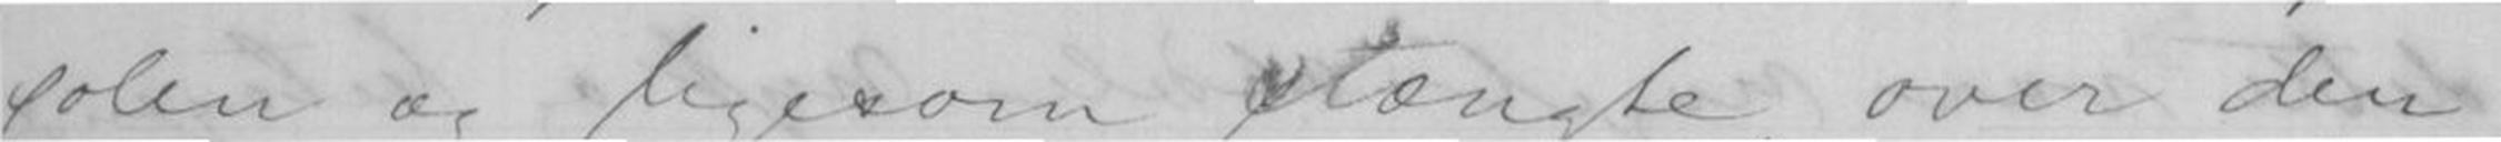solen og ligesom stængte, over den
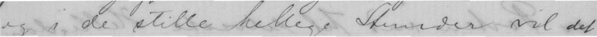og i de stille hellige Stunder vil det
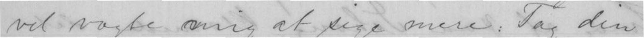vel vogte mig at sige mere: Tag din
